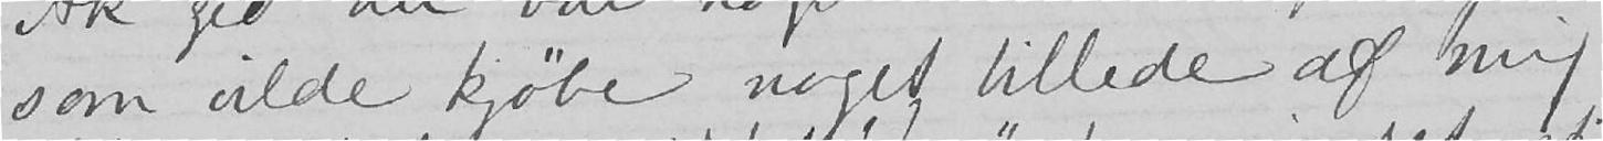som vilde kjøbe noget billede af mig,
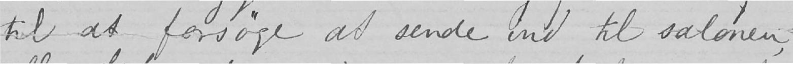til at forsøge at sende ind til salonen,
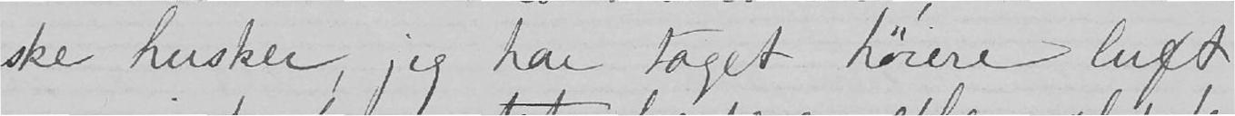ske husker, jeg har taget høiere luft
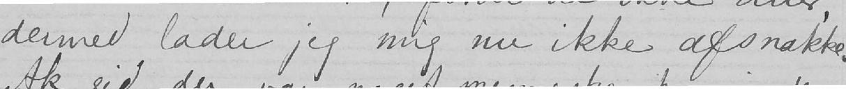dermed lader jeg mig nu ikke afsnakke.
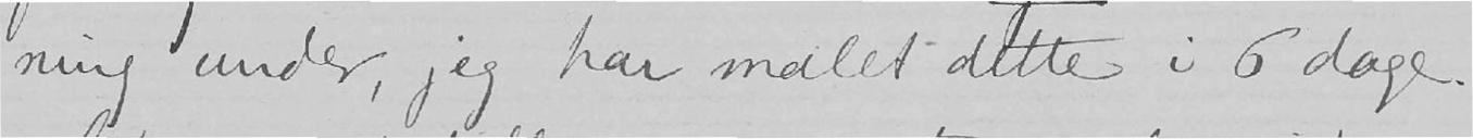ning under, jeg har malet dette i 6 dage.
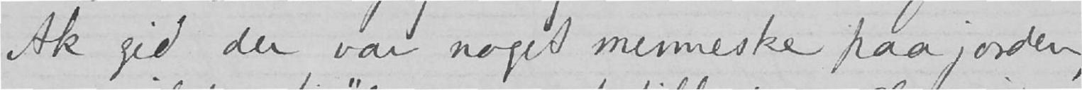Ak gid der var noget menneske paa jorden,
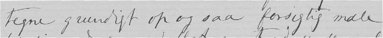tegne grundigt op og saa forsigtig male
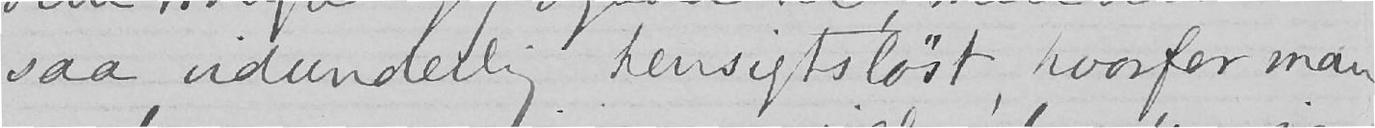saa vidunderlig hensigtsløst, hvorfor man
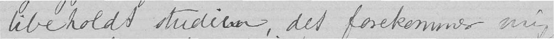bibeholdt studien, det forekommer mig,
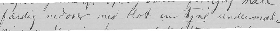færdig nedover med blot en tynd undermal-
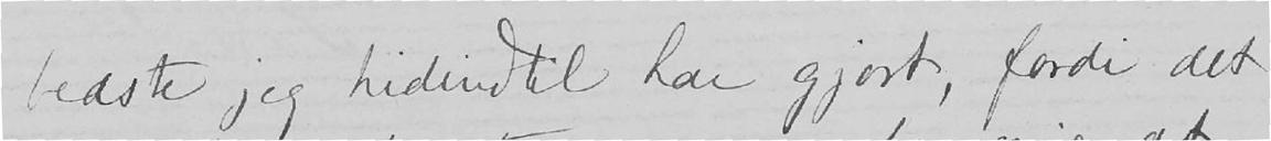bedste jeg hidindtil har gjort, fordi det
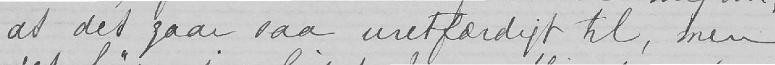at det gaar saa uretfærdigt til, men
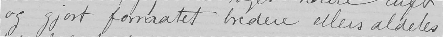og gjort formatet bredere ellers aldeles
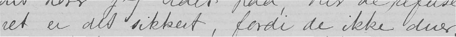ret er det sikkert, fordi de ikke duer,
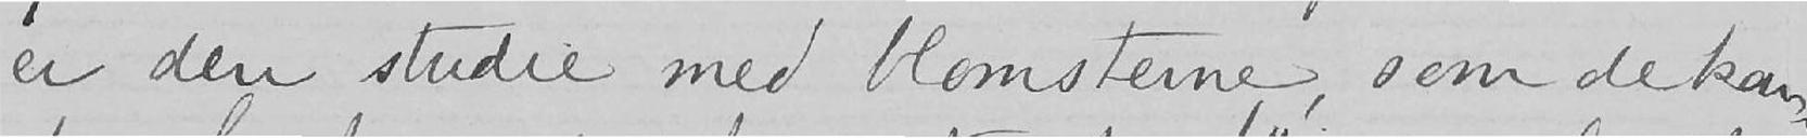er den studie med blomsterne, som de kan-
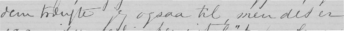dem trængte jeg ogsaa til, men det er
